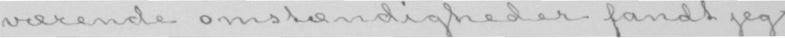værende omstændigheder fandt jeg
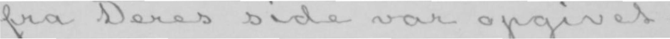fra Deres side var opgivet.
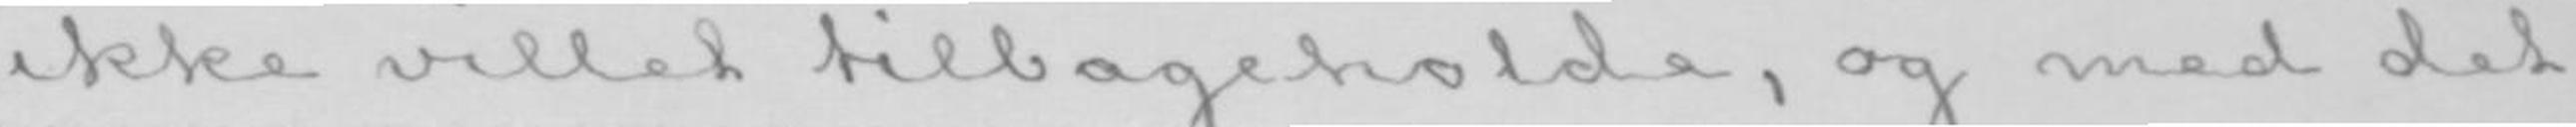ikke villet tilbageholde, og med det
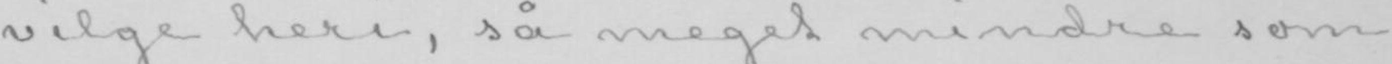vilge heri, så meget mindre som
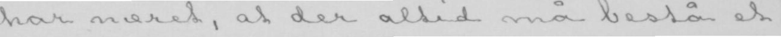har næret, at der altid må bestå et
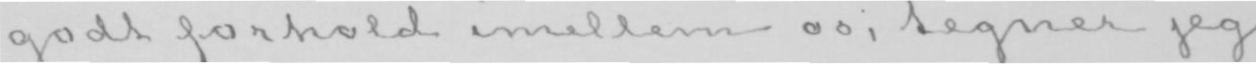godt forhold imellem os, tegner jeg
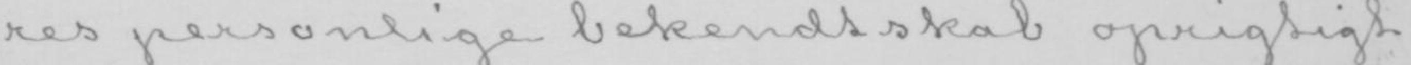res personlige bekendtskab oprigtigt
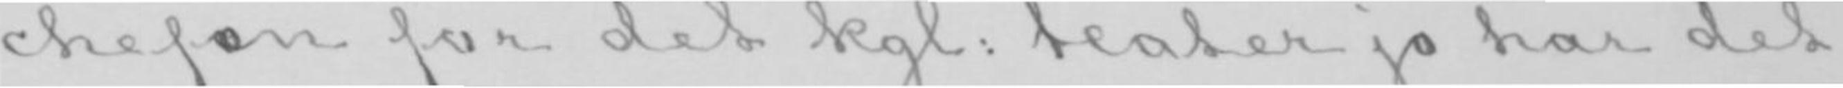chefen for det kgl: teater jo har det
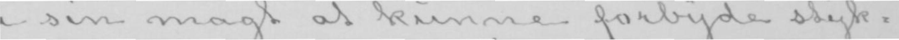i sin magt at kunne forbyde styk-
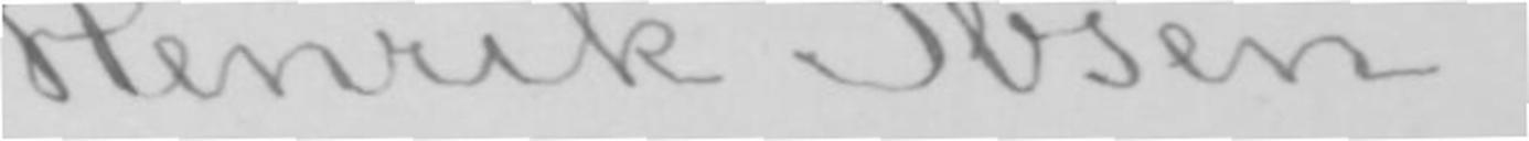Henrik Ibsen.
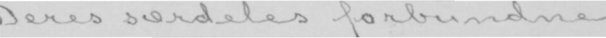Deres særdeles forbundne
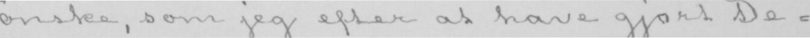ønske, som jeg efter at have gjort De-
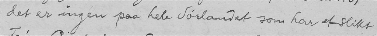det er ingen paa hele Sørlandet som har et slikt
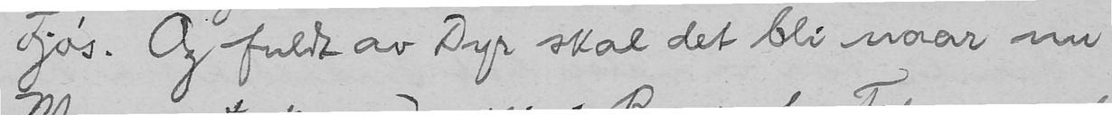Fjøs. Og fuldt av Dyr skal det bli naar nu
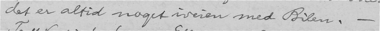det er altid noget iveien med Bilen. -
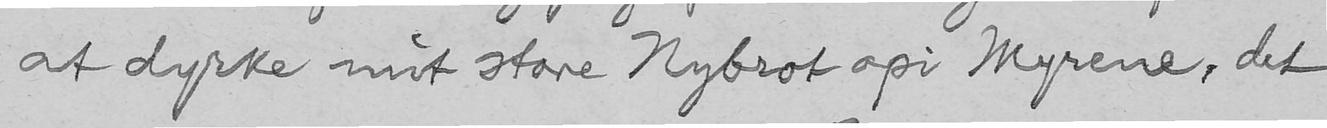at dyrke mit store Nybrot opi Myrene, det
